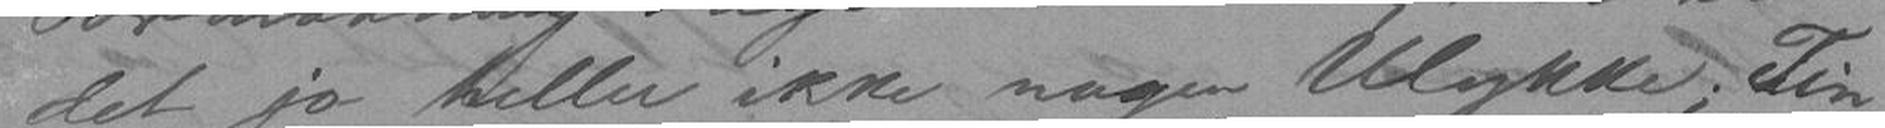det jo heller ikke nogen Ulykke; Tin-
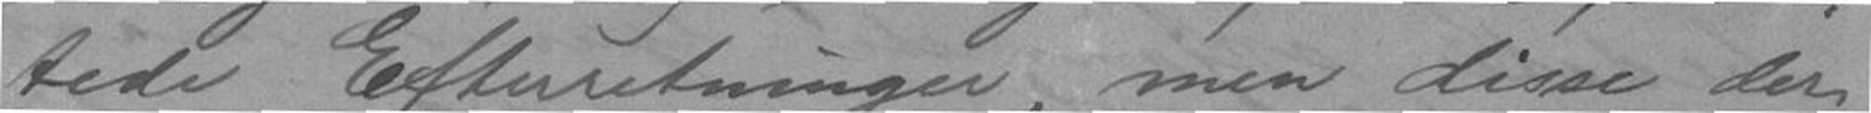tede Efterretninger, men disse deri
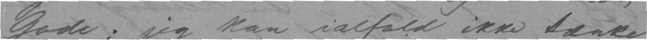Gode; jeg kan ialfald ikke tænke
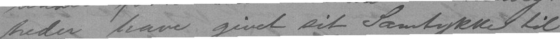heder have givet sit Samtykke til
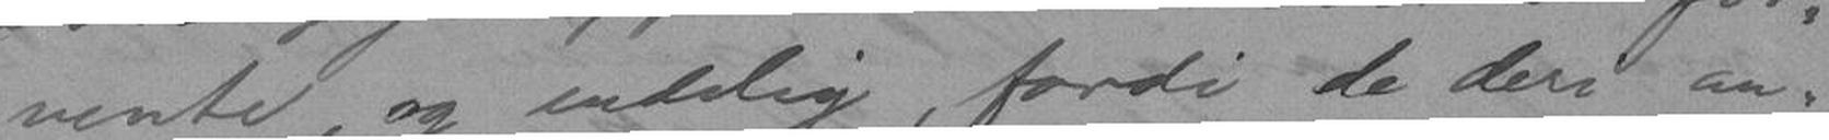vente, og endelig, fordi de deri an-
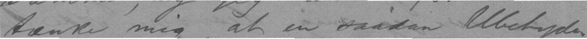tænke mig, at en saadan Ubetyde-
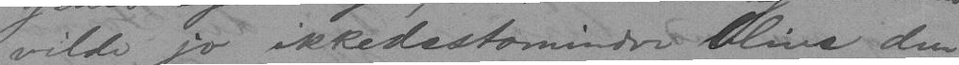vilde jo ikkedestomindre blive den
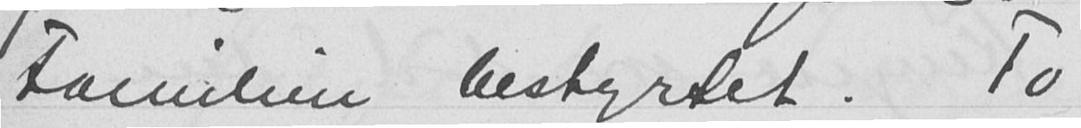Familien bestyrtet. To
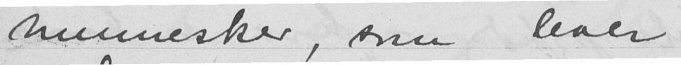mennesker, som lever
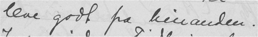leve godt fra hinanden.
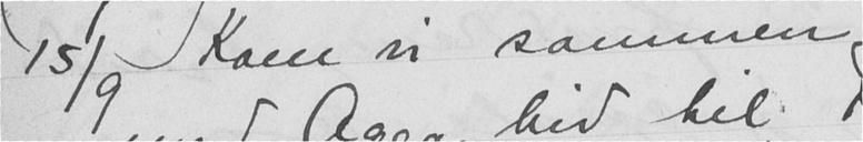15/9 Kom vi sammen
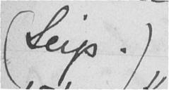(Seip.)
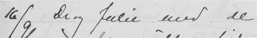16/9 Drog Julie med de
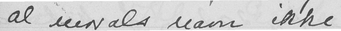al morals navn ikke
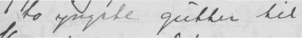to yngste gutter til
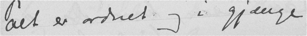alt er ordnet og i gjænge
